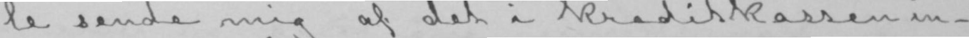le sende mig af det i kreditkassen in-
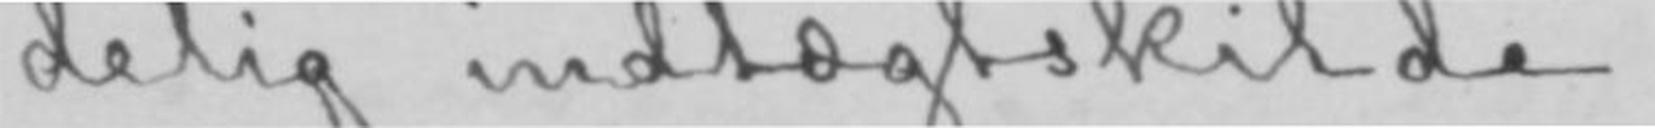delig indtægtskilde.
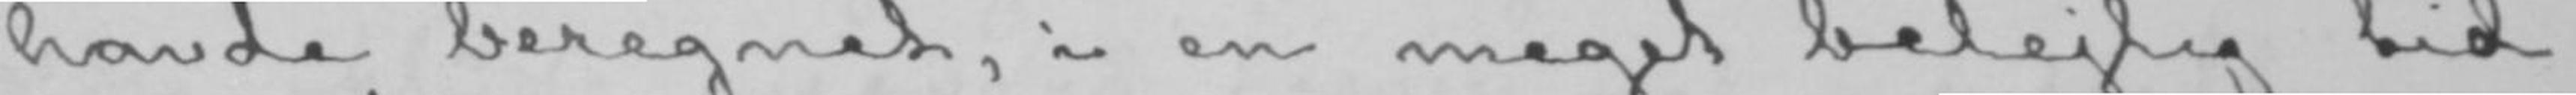havde beregnet, i en meget belejlig tid.
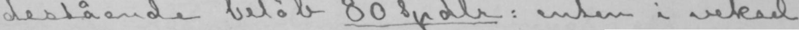destående beløb 80 Spdlr: enten i veksel
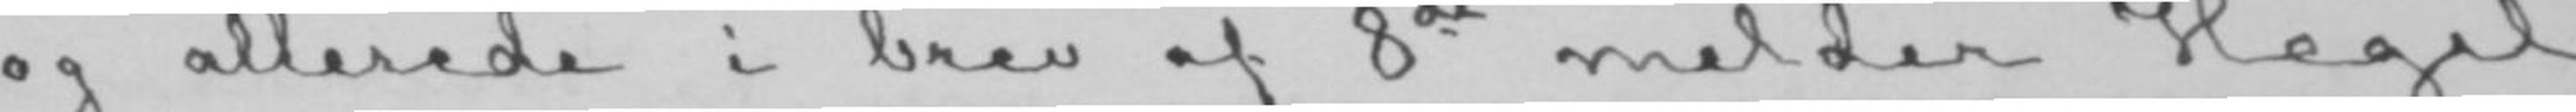og allerede i brev af 8de melder Hegel
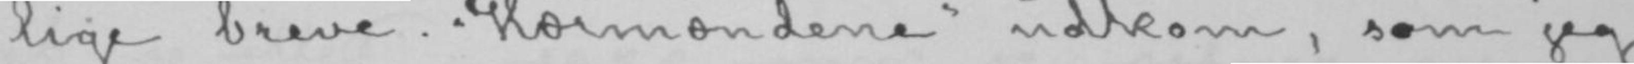lige breve. «Hærmændene» udkom, som jeg
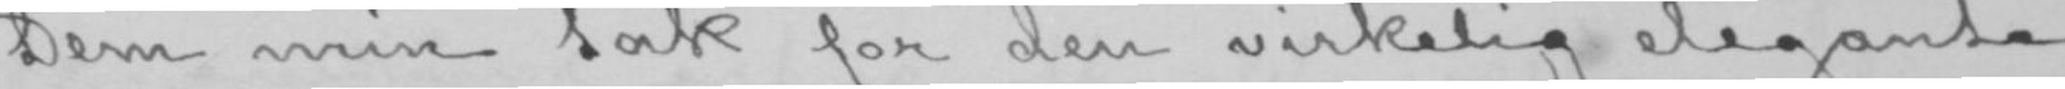Dem min tak for den virkelig elegante
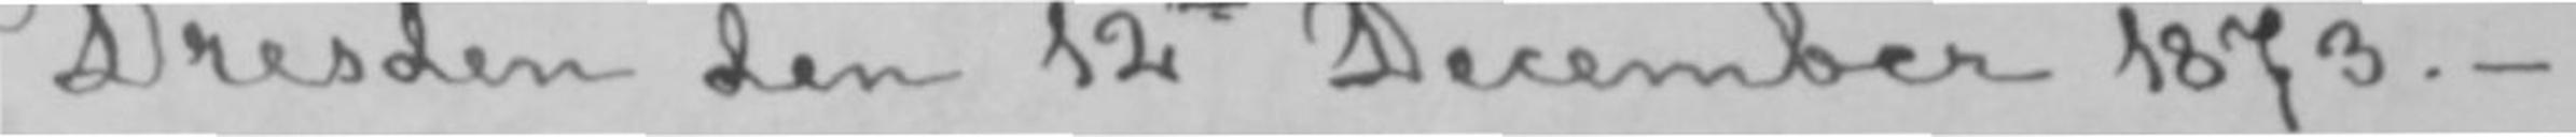Dresden den 12te December 1873.-
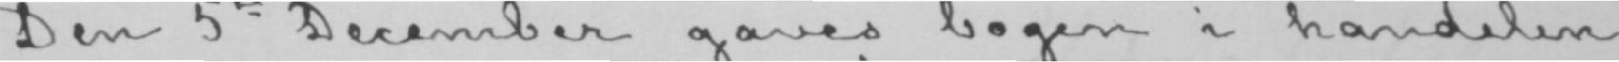Den 5te December gaves bogen i handelen
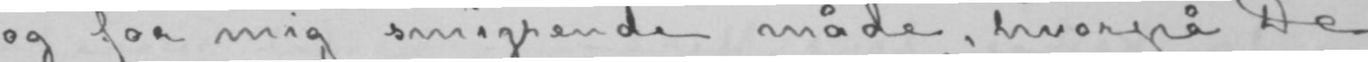og for mig smigrende måde, hvorpå De
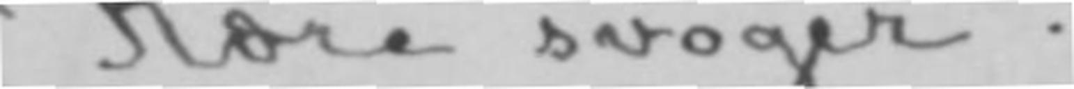Kære svoger!
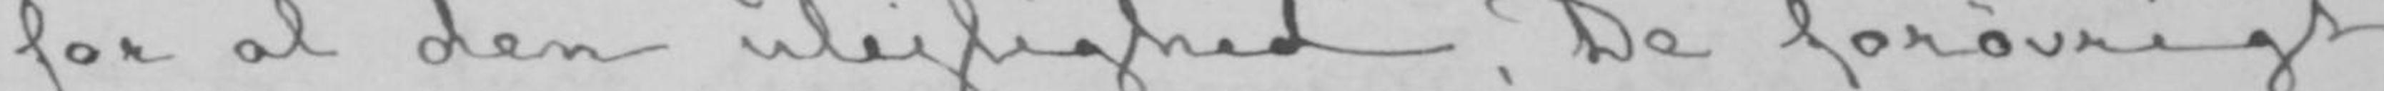for al den ulejlighed, De forøvrigt
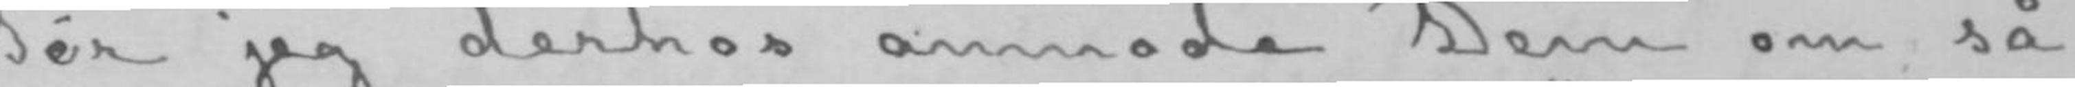Tør jeg derhos anmode Dem om så
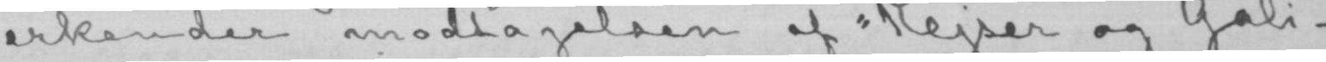erkender modtagelsen af «Kejser og Gali-
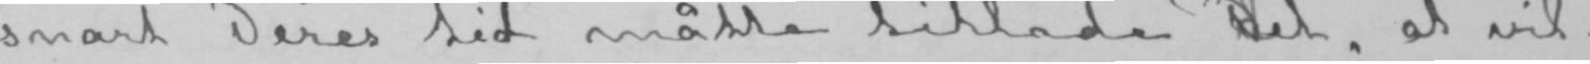snart Deres tid måtte tillade det, at vil-
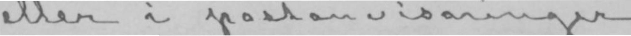eller i postanvisninger.
